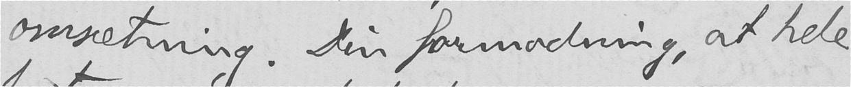omsætning. Din formodning, at hele
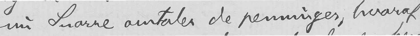nu Snorre omtaler de penninger, hvoraf
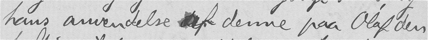hans anvendelse af denne paa Olaf den
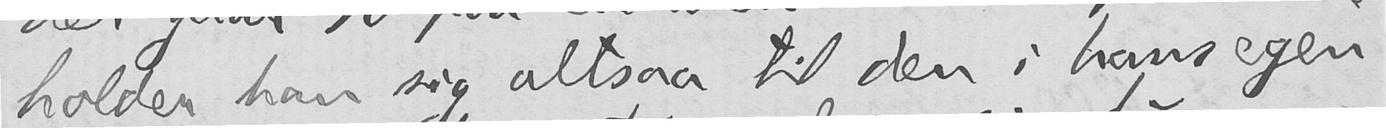holder han sig altsaa til den i hans egen
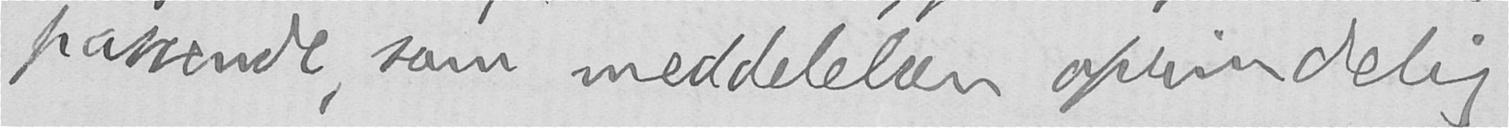passende, som meddelelsen oprindelig
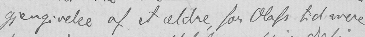gjengivelse af et ældre, for Olafs tid mere
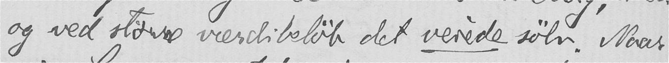og ved større værdibeløb det veiede sølv. Naar
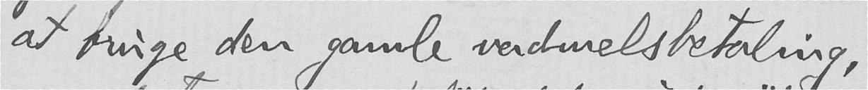at bruge den gamle vadmelsbetaling,
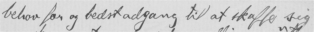behov for og bedst adgang til at skaffe sig
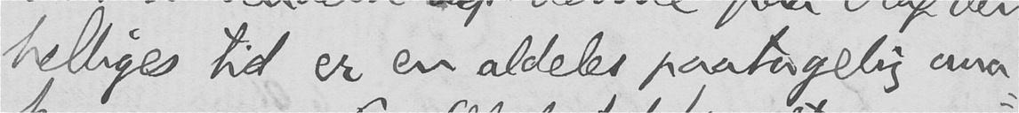helliges tid er en aldeles paatagelig ana-
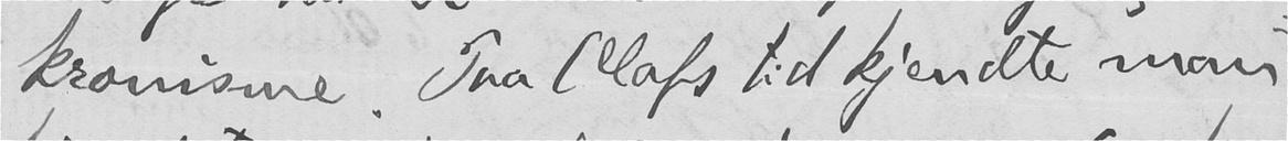kronisme. Paa Olafs tid kjendte man
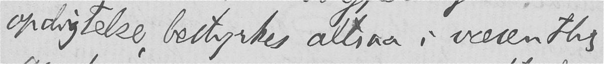opdigtelse, bestyrkes altsaa i væsentlig
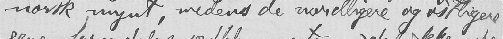norsk mynt, medens de nordligere og østligere
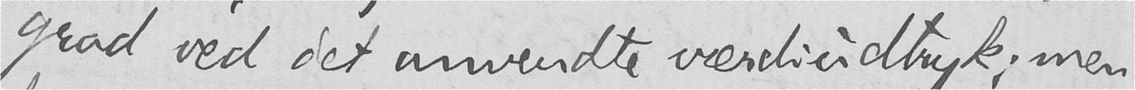grad ved det anvendte værdiudtryk; men
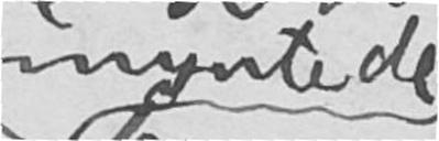myntede
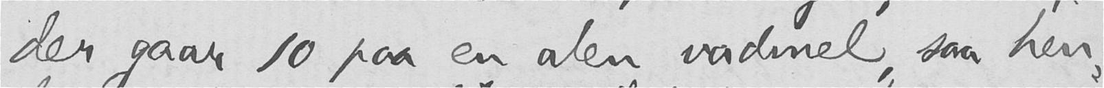der gaar 10 paa en alen vadmel, saa hen-
